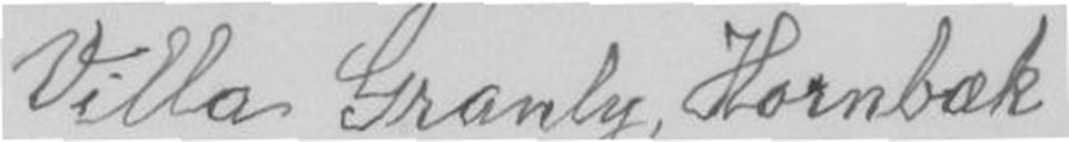Vila Granly, Hornbæk
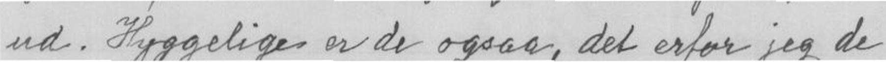ud. hyggelig er de ogsaa, det erfor jeg de
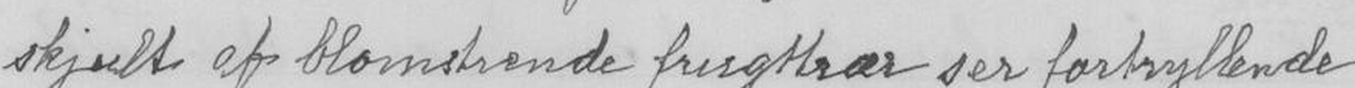skjult af blomstrende frugttrær ser fortryllende
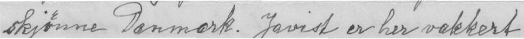skjønne Dænmærk. Javist er her vakkert
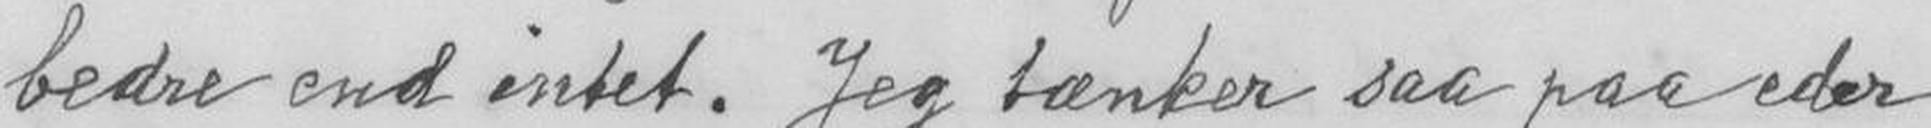bedre end intet. jeg tænker saa paa eder
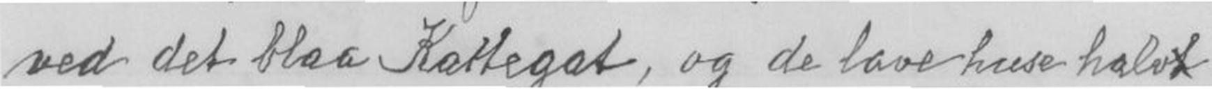ved det blaa Kattegat, og de lave huse halvt
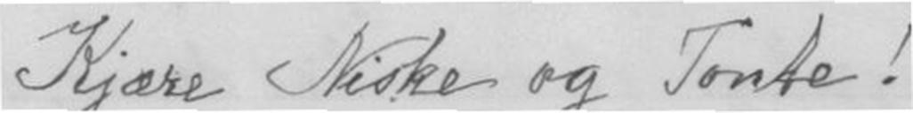Kjære Niske og Tonte!
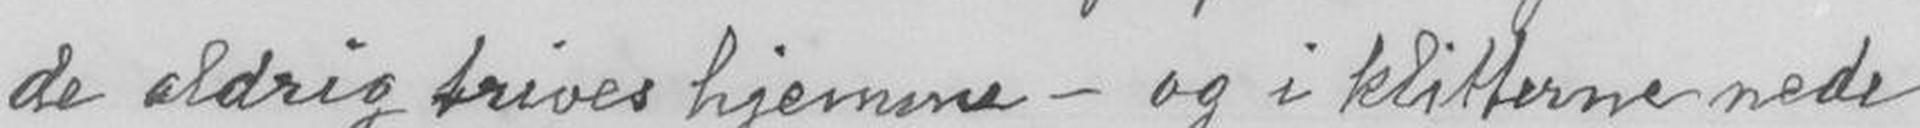de aldrig trives hjemme - og i klitterne nede
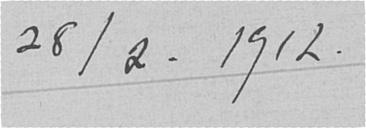28/2-1912.
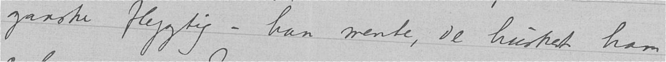ganske flygtig – han mente, de husket ham
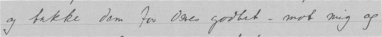og takke dem for Deres godhet – mot mig og
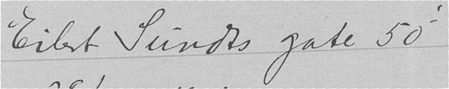Eilert Sundts gate 50I
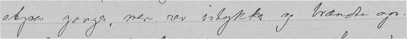etpar ganger, men rev istykker og brændte op.
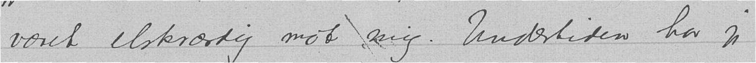været elskværdig mot mig. Undertiden har jeg
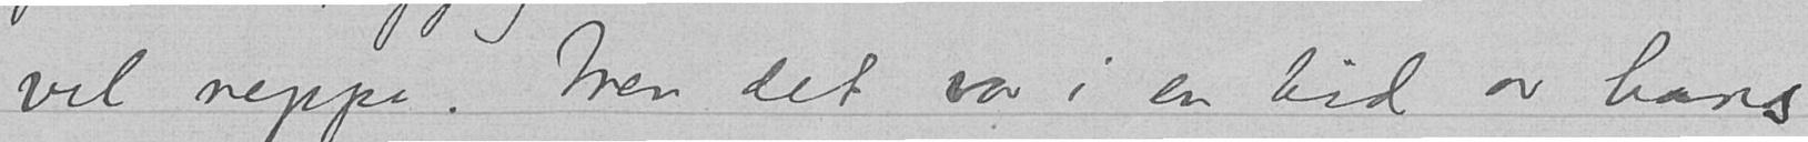vel neppe. Men det var i en tid av hans
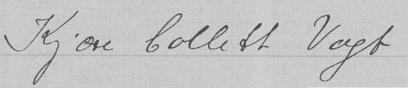Kjære Collett Vogt
